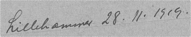Lillehammer 28.11.1919.
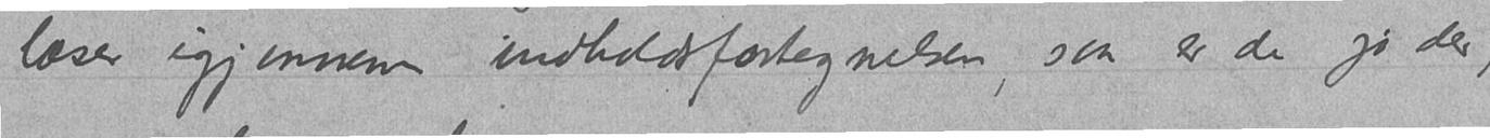læser igjennem indholdsfortegnelsen, saa er de jo der,
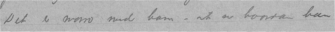Det er morro med ham – at se hvordan han
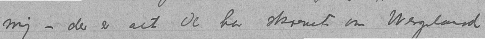mig – der er alt De har skrevet om Wergeland
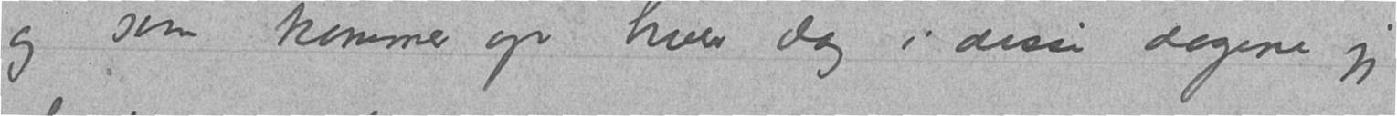og som kommer op hver dag i disse dagene jeg
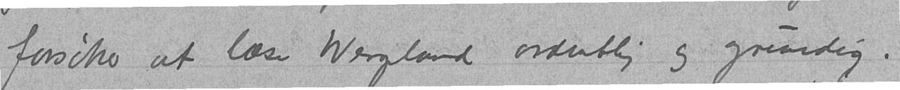forsøker at læse Wergeland ordentlig og grundig.
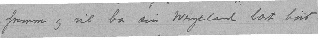fremme og vil ha sin Wergeland lært høit.
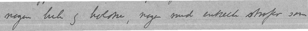nogen hele og holdne, nogen med enkelte strofer som
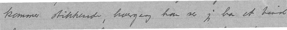kommer stikkende, hvergang han ser jeg har et bind
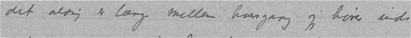det aldrig er længe mellem hvergang jeg hører indi
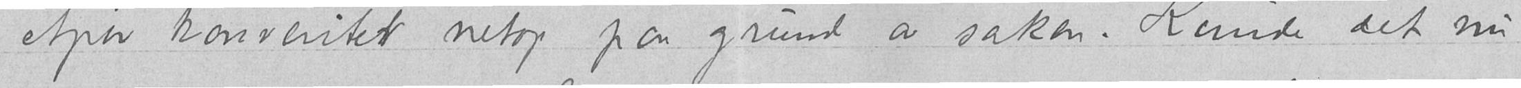etpar konveriter netop paa grund av saken. Kunde det nu
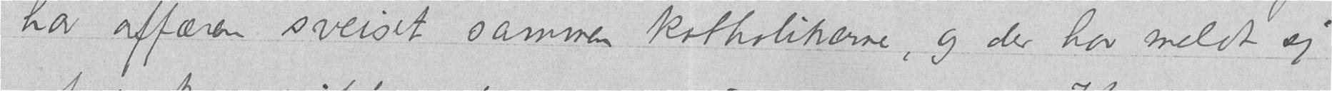har affæren sveiset sammen katholikerne, og der har meldt sig
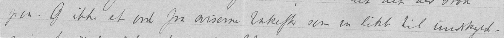paa. Og ikke et ord fra aviserne bakefter som var likt til undskyld-
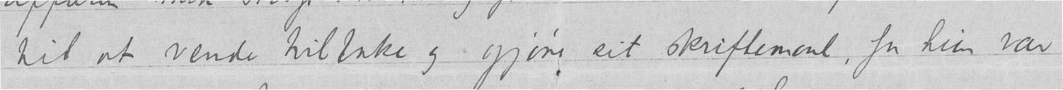til at vende tilbake og gjøre sit skriftemaal, for hun var
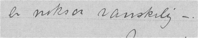er noksåa vanskelig –.
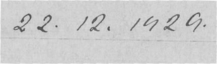22.12.1929.
